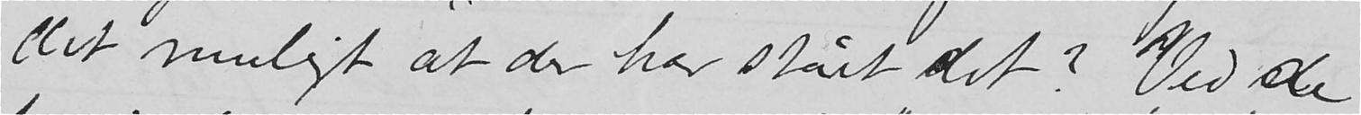det muligt at der har stået det? Ved de
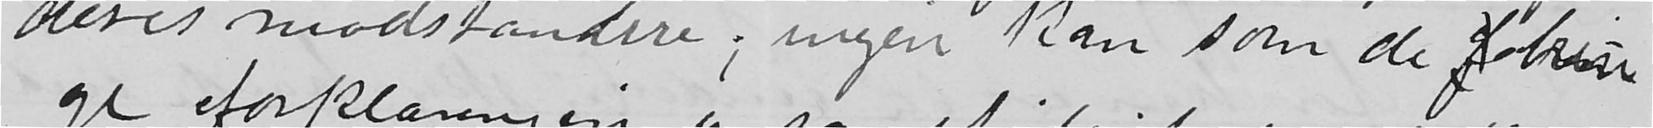deres modstandere; ingen kan som de fo brin-
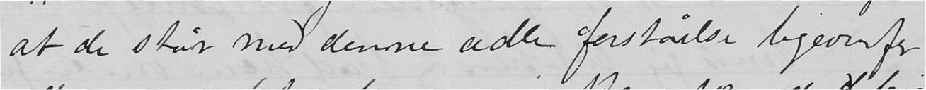at de står med denne ædle forståelse ligeoverfor
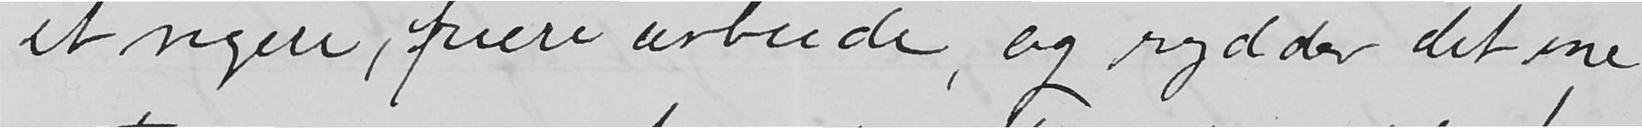et rigere, friere arbeide, og rydder det ene
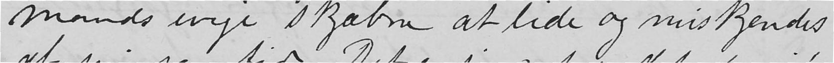mænds evige skjæbne at lide og miskjendes
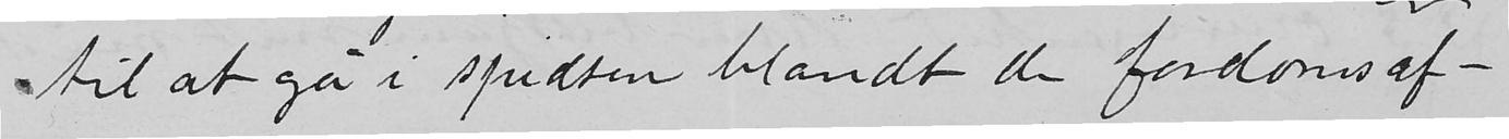til at gå i spidsen blandt de fordomsaf-
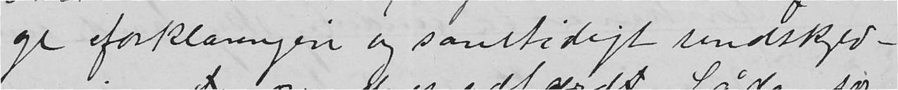ge forklaringen og samtidigt undskyld-
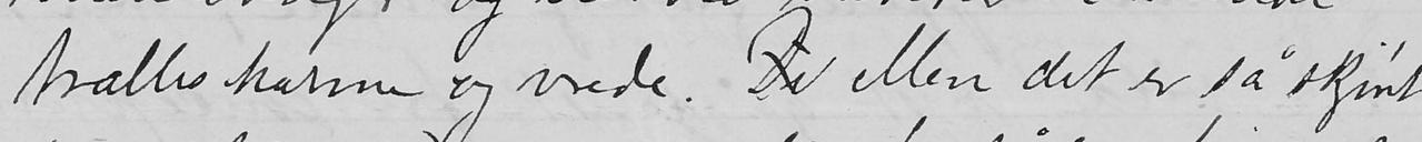trælles harme og vrede. De Men det er så skjønt
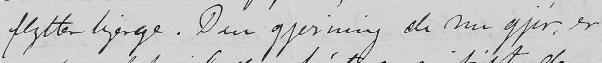flytter bjerge. Den gjerning de nu gjør, er
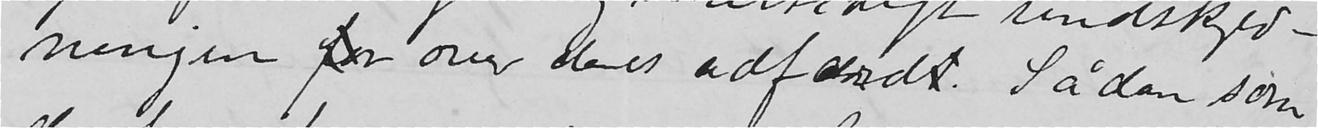ningen for over deres adfærdt. Sådan som
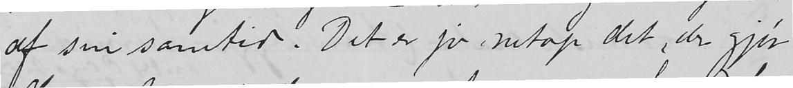af sin samtid. Det er jo netop det, der gjør
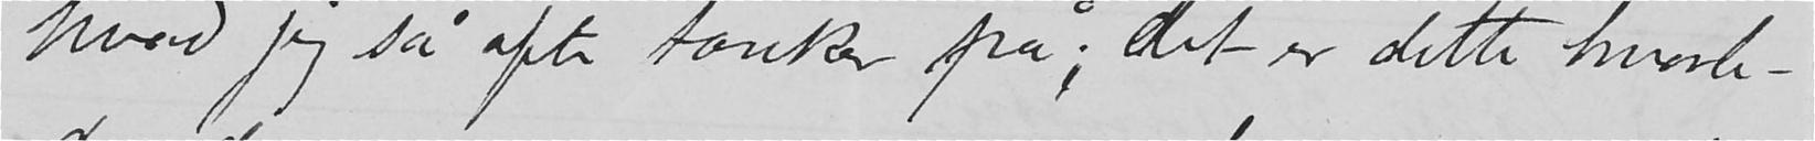hvad jeg så ofte tænker på; det er dette hvorle-
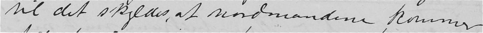vil det skyldes, at nordmændene kommer
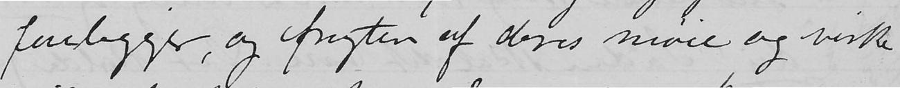foreligger, og frugten af deres møie og virke
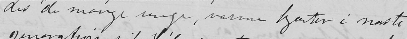des de mange unge, varme hjærter i næste
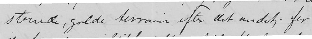stenede, golde terrain efter det andet, for
